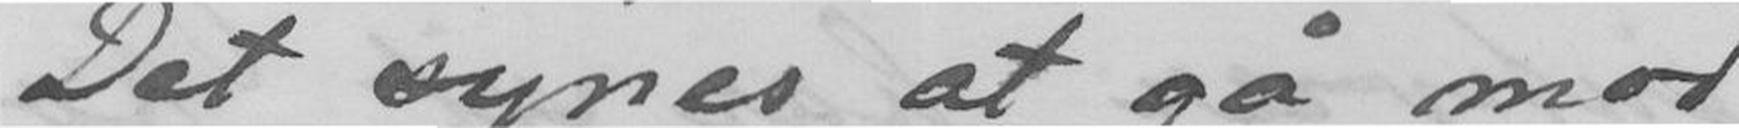Det synes at gå mod
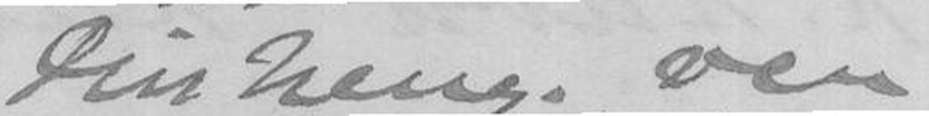Din heng. ven
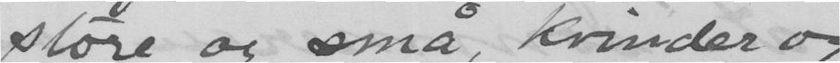store og små, kvinder og
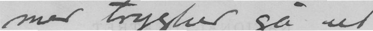med tryghed gå ud
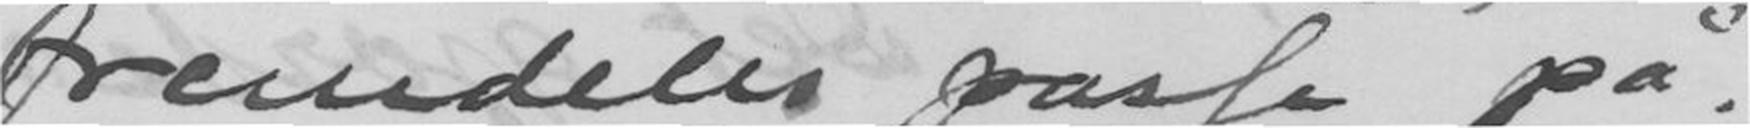fremdeles passe på.
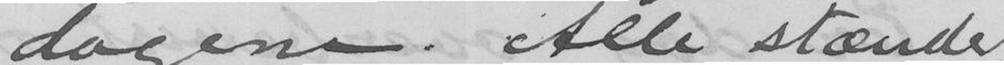dagene. Alle stænde,
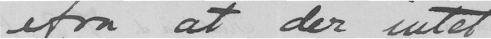ifra at der intet
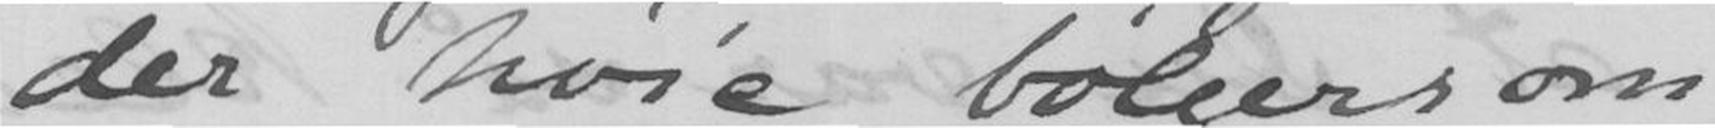der høie bølger om
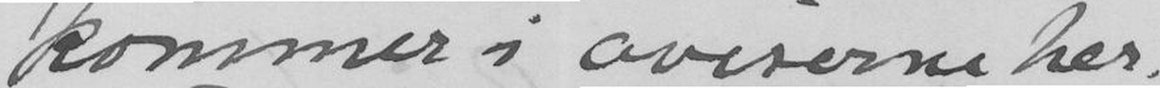kommer i aviserne her.
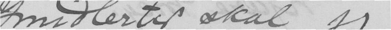Imidlertid skal jeg
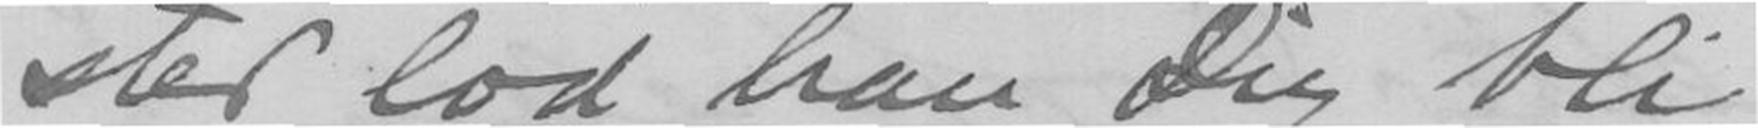sted lod han Dig bli
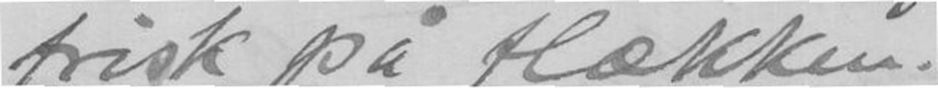frisk på flækken.
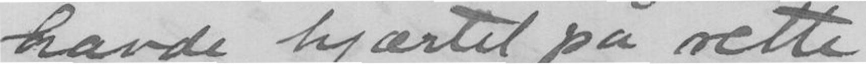havde hjærtet på rette
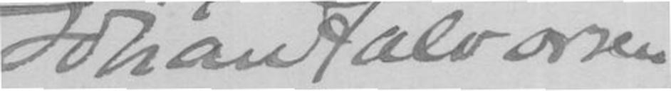Johan Halvorsen
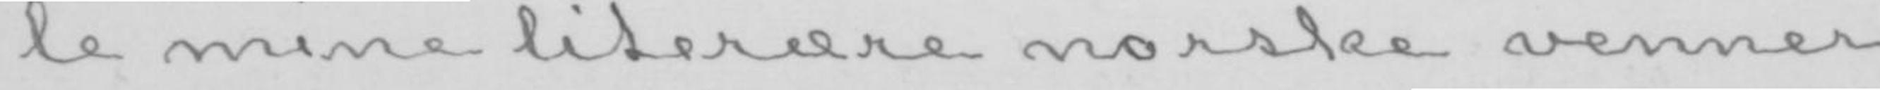le mine literære norske venner
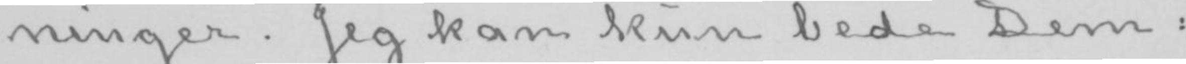ninger. Jeg kan kun bede Dem:
